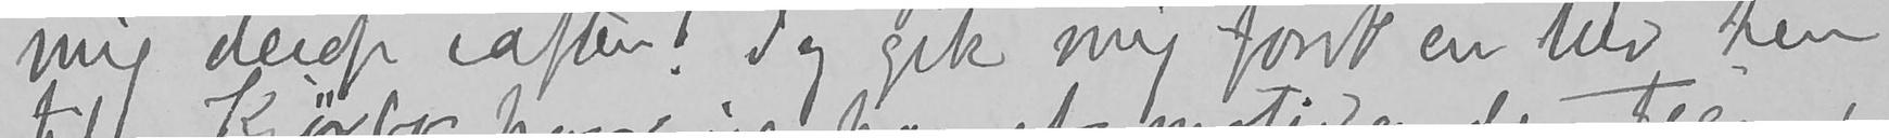mig derop iaften. Jeg gik mig først en tur hen
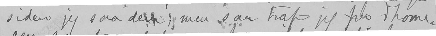siden jeg saa dem; men saa traf jeg fru Thome-
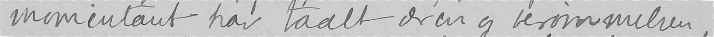momentant har taalt æren og berømmelsen,
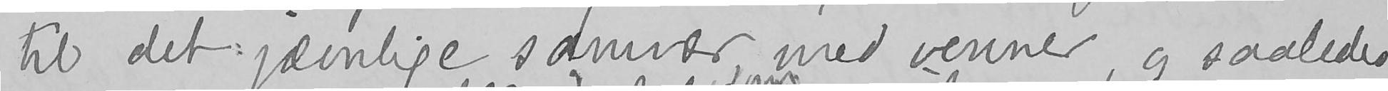til det jævnlige samvær med venner, og saaledes
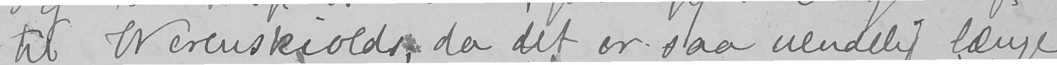til Werenskiolds, da det er saa uendelig længe
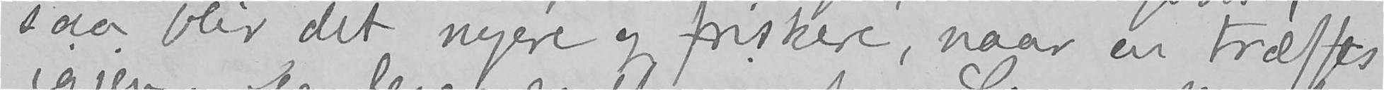saa blir det nyere og friskere, naar en træffes
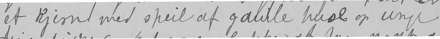et Kjern med speil af gamle huse og unge
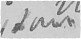som
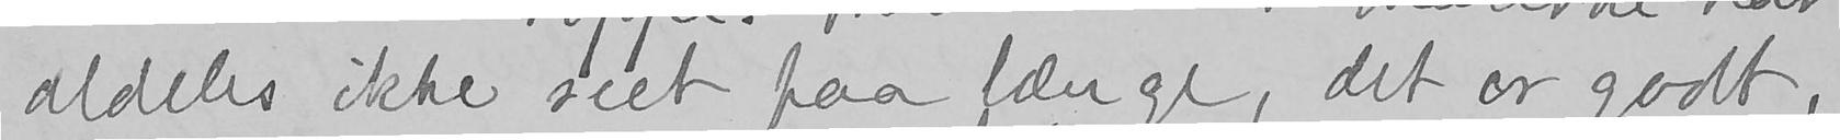aldeles ikke seet paa længe, det er godt,
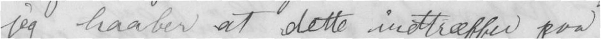jeg haaber at dette indtræffer paa
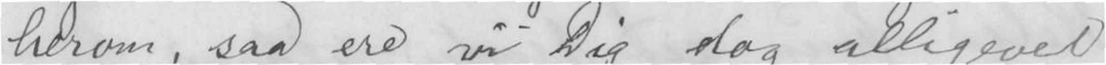herom, saa ere vi Dig dog alligevel
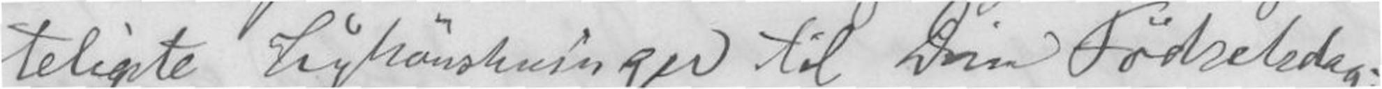teligste Lykønskninger til Din Fødselsdag;
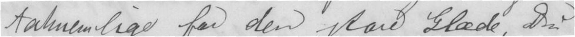taknemlige for den store Glæde, Du
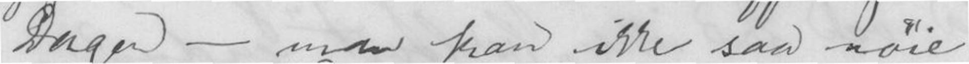Dagen - man kan ikke saa nøie
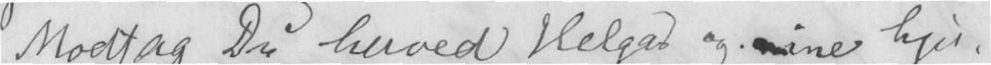Modtag Du herved Helgas og mine hjer-
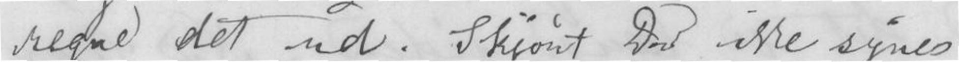regne det ud. Skjønt Du ikke synes
